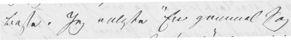Beste. Jeg valgte "En gammel Sag
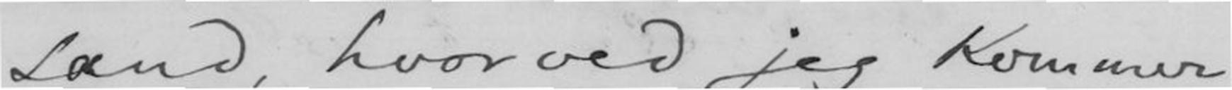sand, hvor ved jeg Kommer
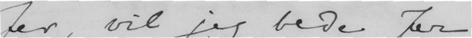Jer, vil jeg bede Jer
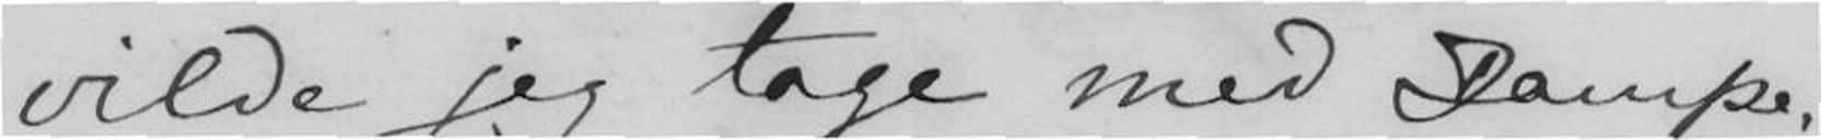vilde jeg tage med Dampe-
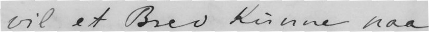vil et Brev kunne naa
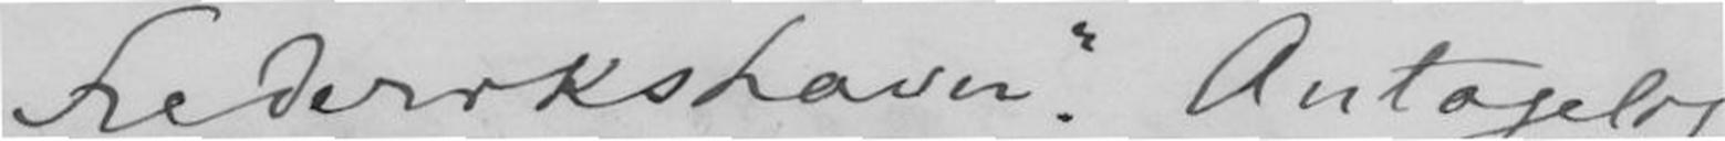Frederikshavn Antagelig
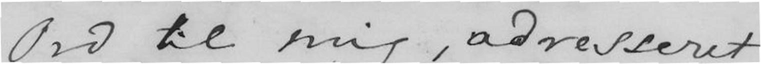Ord til mig, adresseret
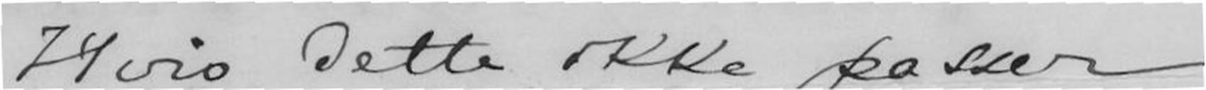Hvis dette ikke passer
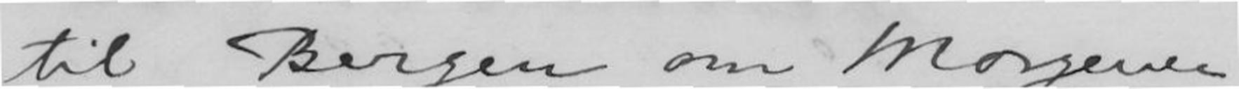til Bergen om Morgenen
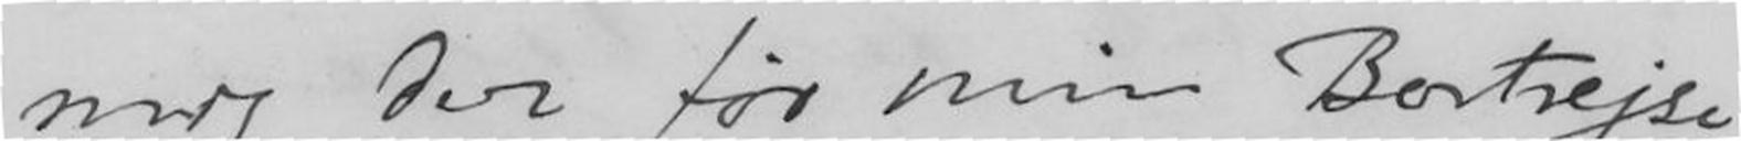mig der før min Bortrejse
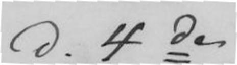d. 4de.
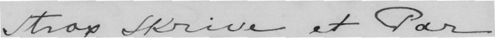strax skrive et Par
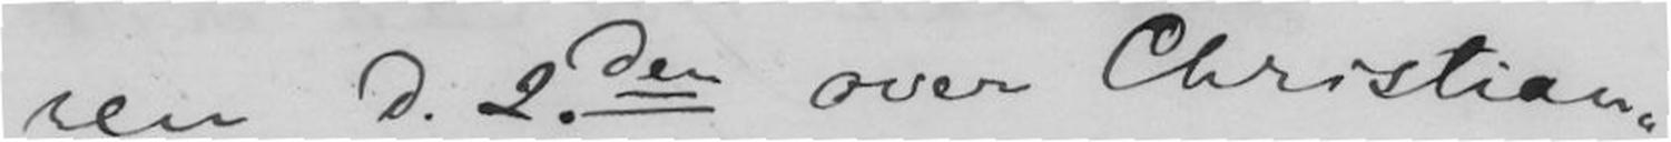ren d. 2.den over Christian-
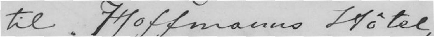til J Hoffmanns Hotel,
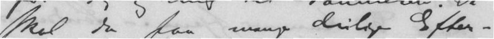skal da faa mange deilige Efter-
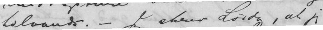tilvands. - Jeg skrev Lørdag, at jeg
